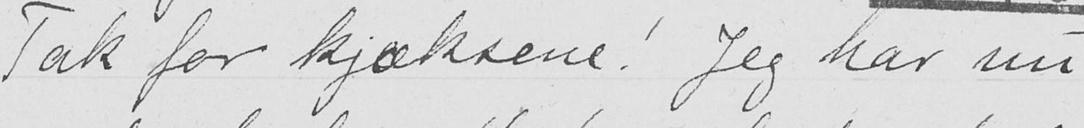Tak for kjæksene! Jeg har nu
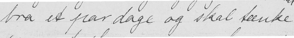bra et par dage og skal tænke
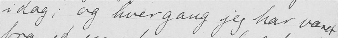idag; og hver gang jeg har været
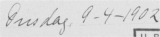Onsdag, 9-4-1902
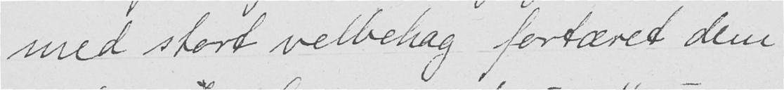med stort velbehag fortæret dem
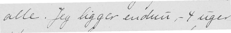alle. Jeg ligger endnu, - 4 uger
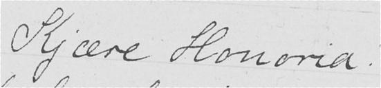Kjære Honoria!
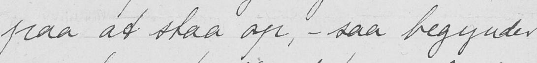paa at staa op, - saa begynder
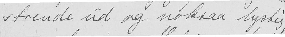strende ud og noksaa lystig
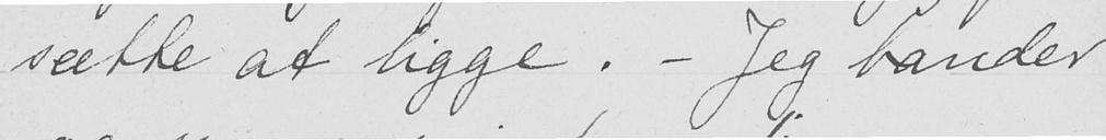sætte at ligge. - Jeg bander
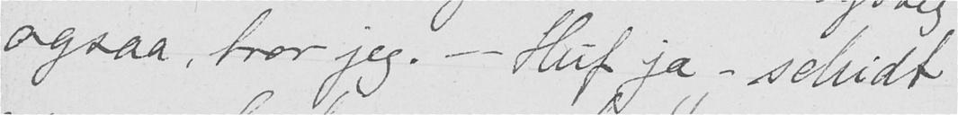ogsaa, tror jeg. -- Huf ja - schidt
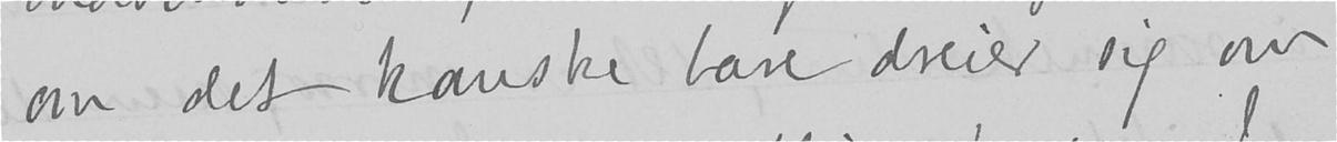om det kanske bare dreier sig om
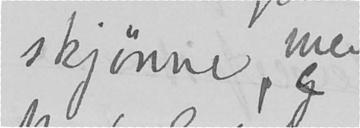skjønne, og
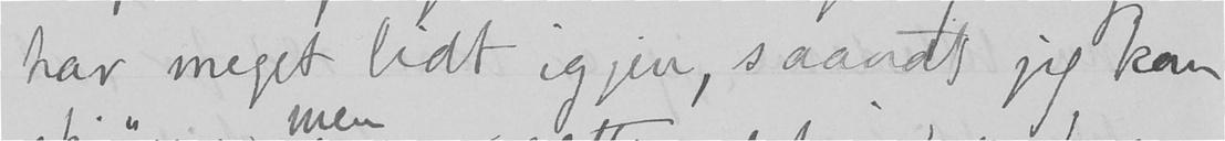har meget lidt igjen, saavidt jeg kan
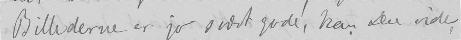Billederne er jo svært gode, kan Du vide,
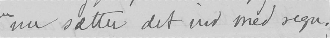nu sætter det ind med regn.
